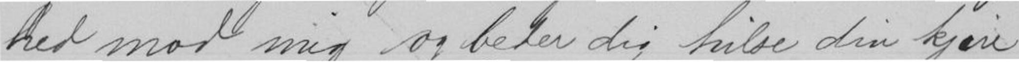hed mod mig og beder dig hilse din kjære
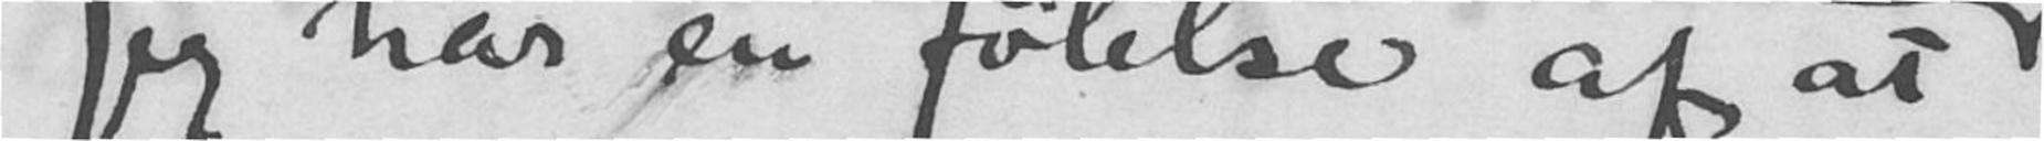jeg har en følelse af at
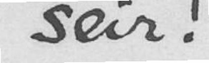seir!
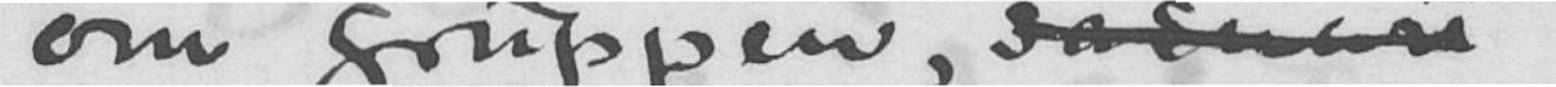om gruppen, så snart
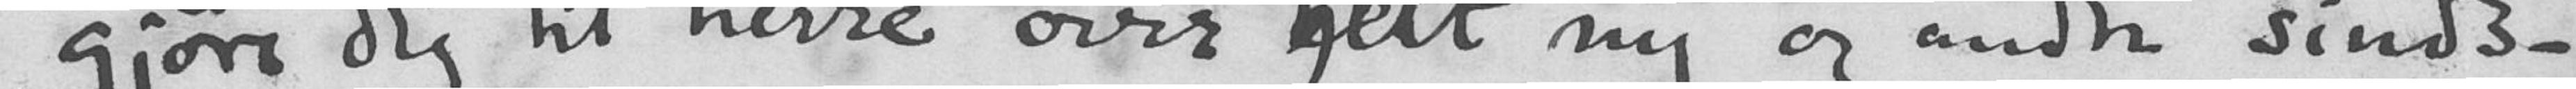gjøre dig til herre over helt ny og andre sinds-
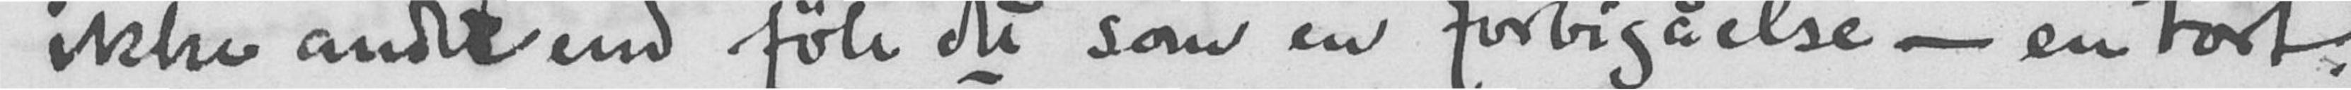ikke andet end føle det som en forbigåelse - en tort.
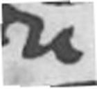du i
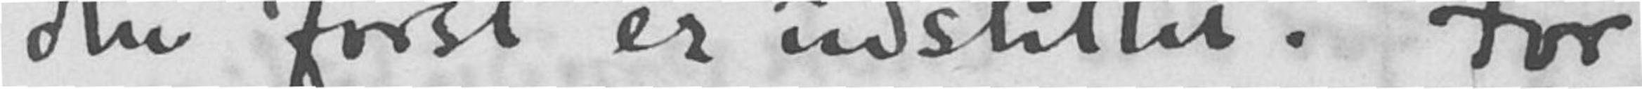den først er udstillet. Før
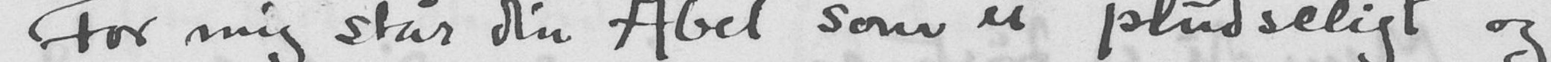For mig står din "Abel" som et pludseligt og
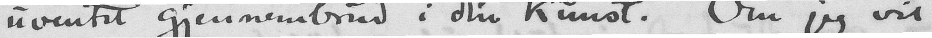uventet gjennembrud i din kunst. Om jeg vil
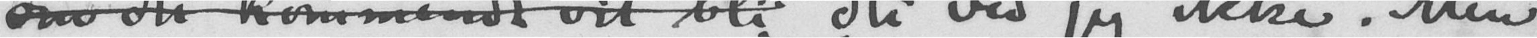om det kommende vil bli det ved jeg ikke. Men
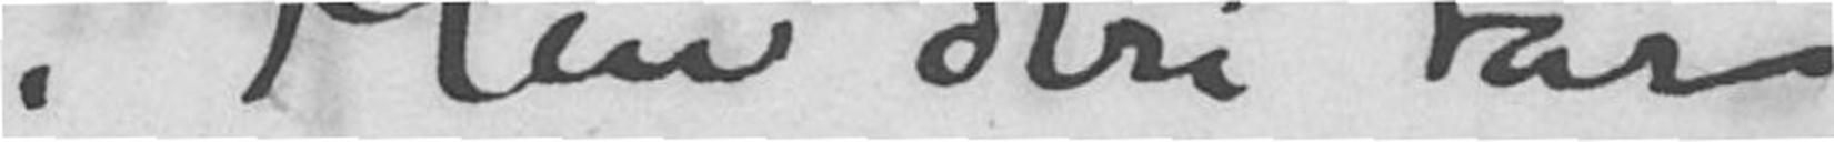. Men deri tar
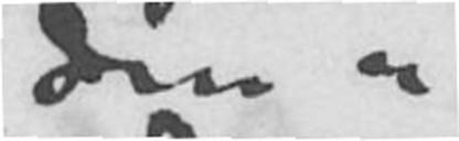din
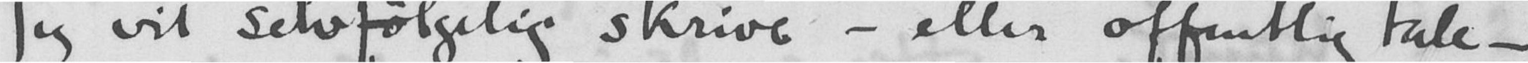Jeg vil selvfølgelig skrive - eller offentlig tale -
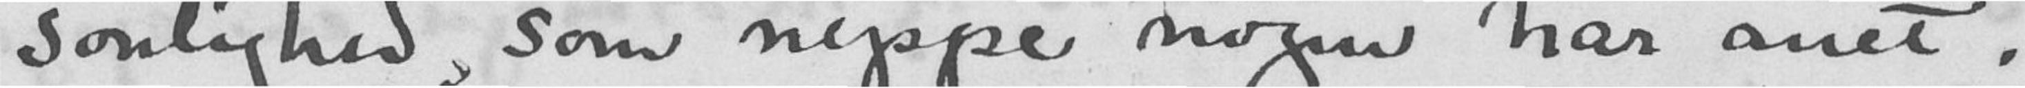sonlighed, som neppe nogen har anet.
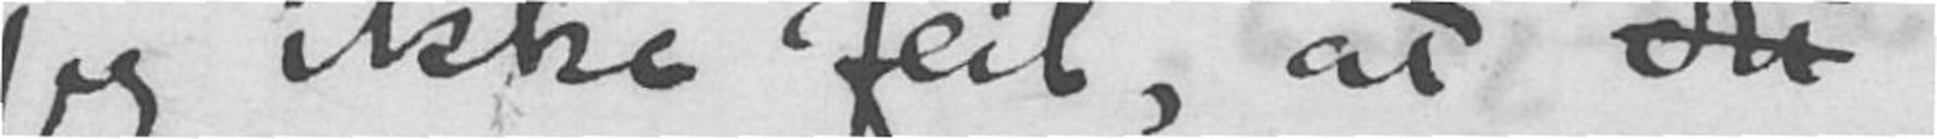jeg ikke feil, at du
